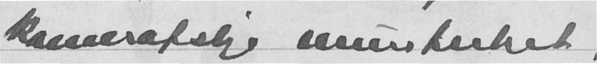komerafslye miitirket
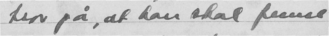tror på, at han skal finne
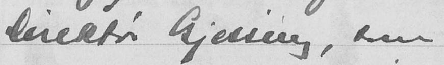Direktør Gjessing, som
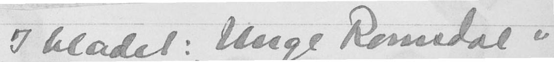I bladet: "Unge Romsdal"
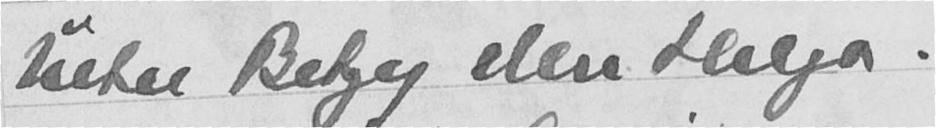blter Behy elere Helga.
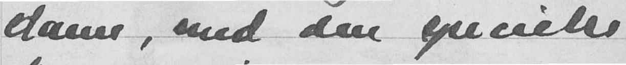dame, med den specielle
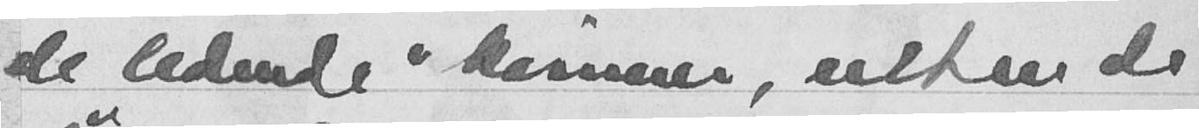de ledende kvinner, uten de
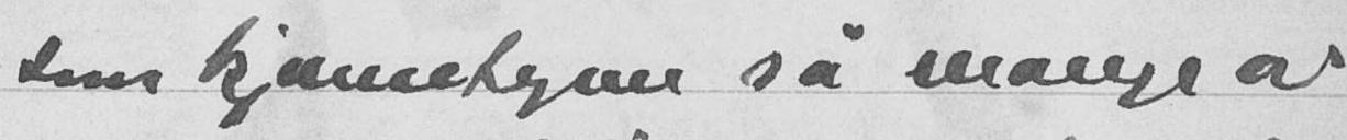som kjennetegner så mange av
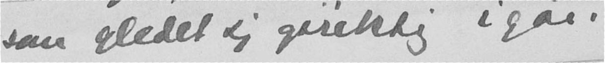som gledet sig opriktig i går.
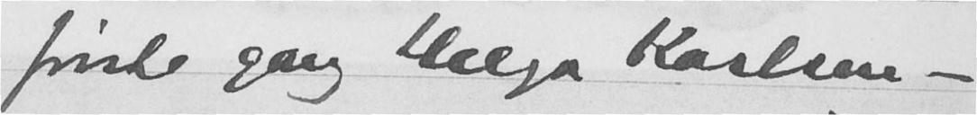første gang Helga Karlsen -
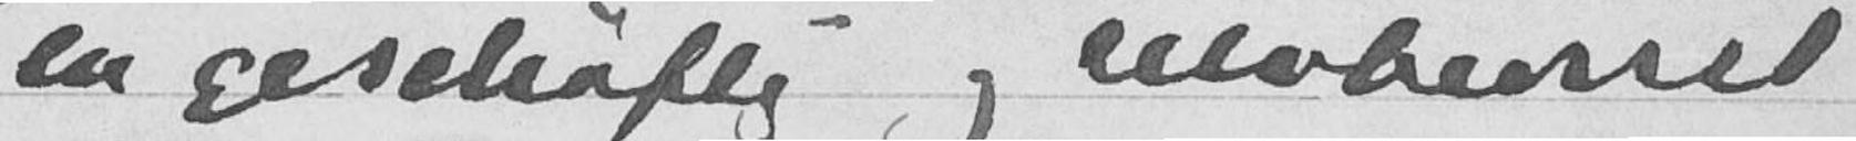en geseliåflig og selvbevisst
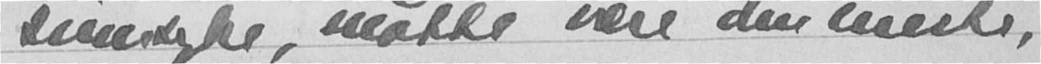sinsyke, måtte være den eneste,
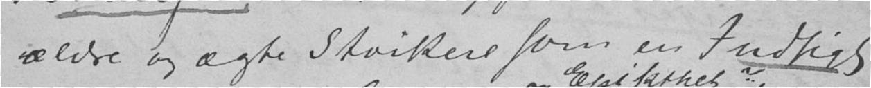ældre og ægte Stoikere som en Indsigt.
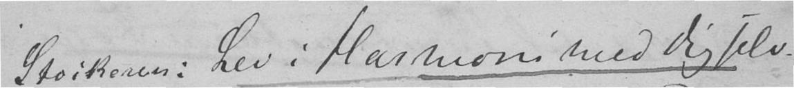Stoikeren: Lev i Harmoni med dig selv
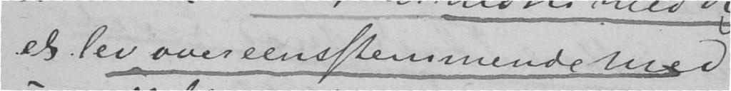el lev overeensstemmende med
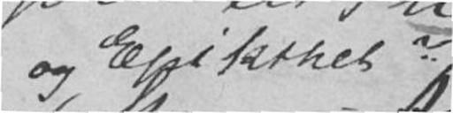og Epikthet?
